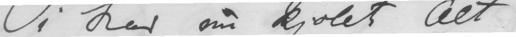Vi har nu kjøbt Alt
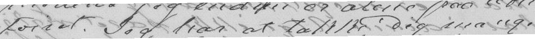toiret. Jeg har at takke Dig mange
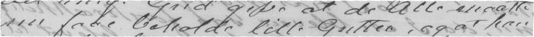nu faae beholde lille Gutten, og at han
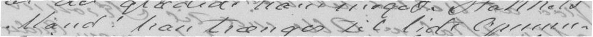Mand! han trænger til lidt Opmun-
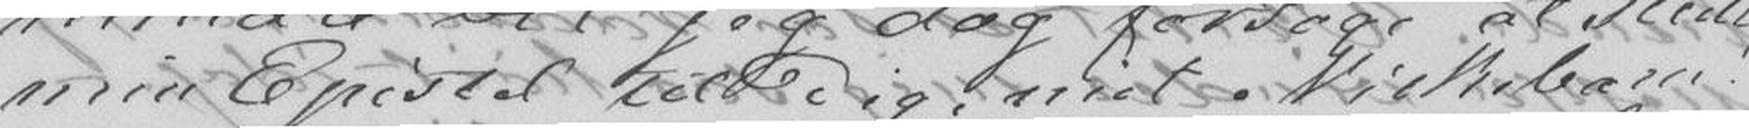min Epistel til Dig, mit Niskebarn!
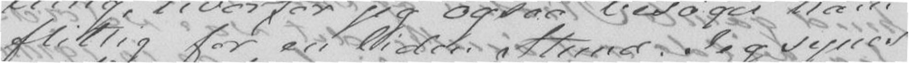flittig for en liden Stund. Jeg synes
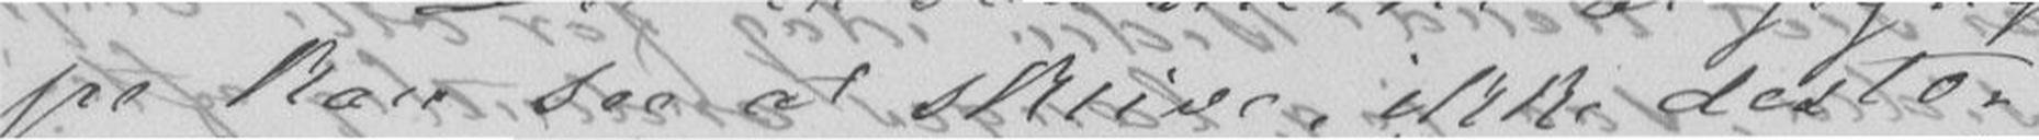pe kan see at skrive, ikke desto-
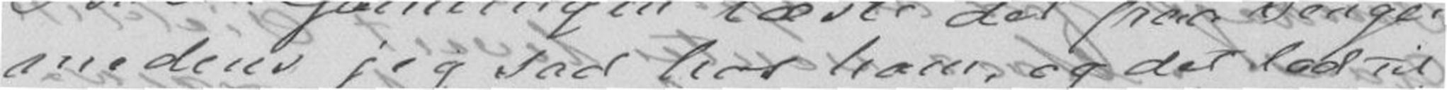medens jeg sad hos ham, og det lod til
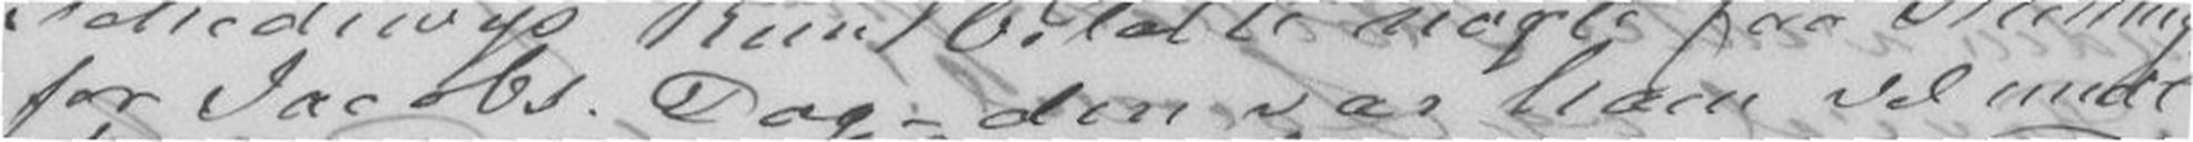for Jacobs. Dog - den var ham vel undt
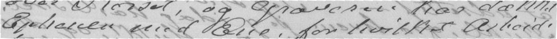Ephaven med Ene, for hvilket Arbeide
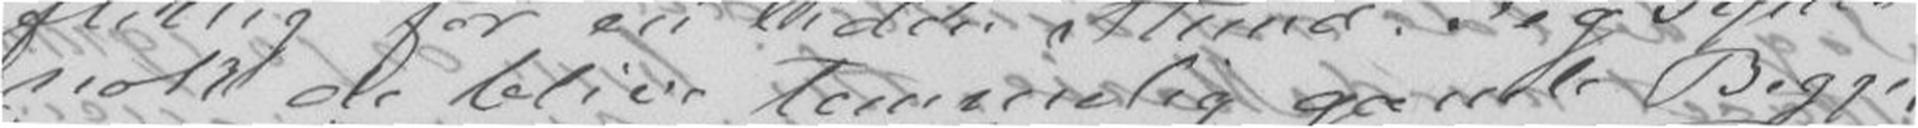nok de blive temmelig gamle Begge,
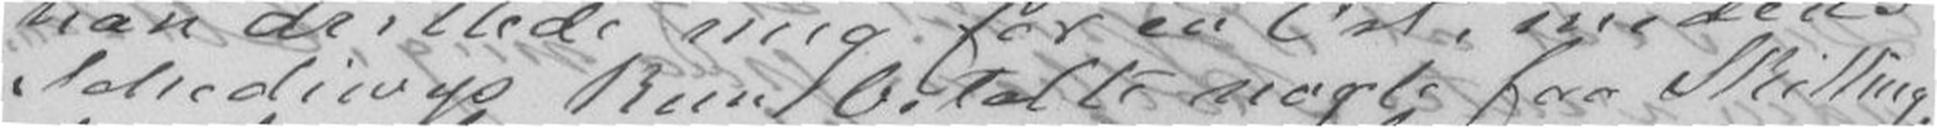Schediwys kun betalte nogle faa Skilling
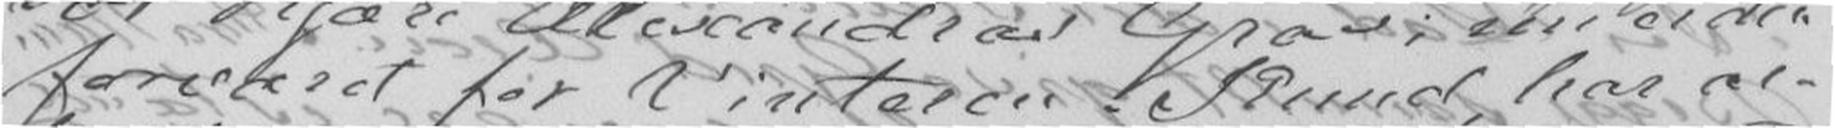forværet for Vinteren. Knud har ar-
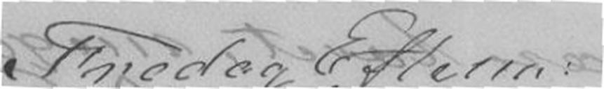Fredag Efterm:
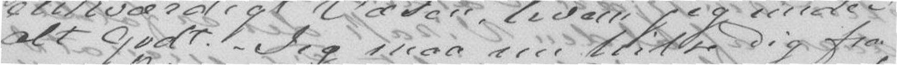alt godt. Jeg maa nu hilse Dig fra
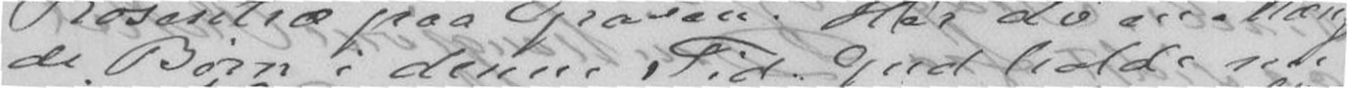de Børn i denne Tid. Gud holde nu
